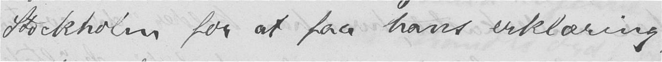Stockholm for at faa hans erklæring,
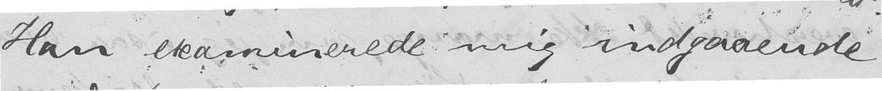Han examinerede mig indgaaende
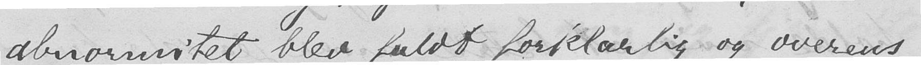abnormitet blev fuldt forklarlig og overens-
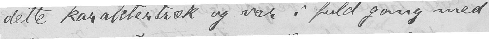dette karaktertræk og var i fuld gang med
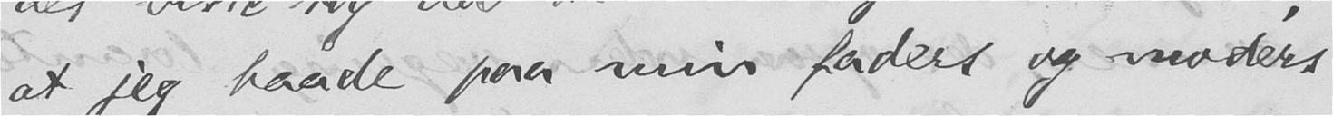at jeg baade paa min faders og moders
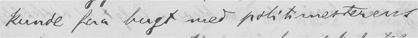kunde faa bugt med politimesterens
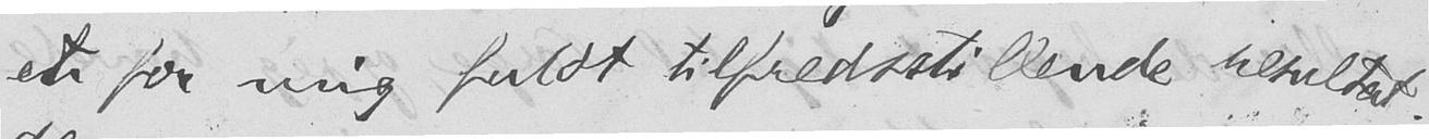et for mig fuldt tilfredsstillende resultat.
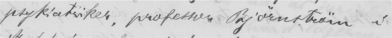psykiatiker, professor Bjørnstrøm i
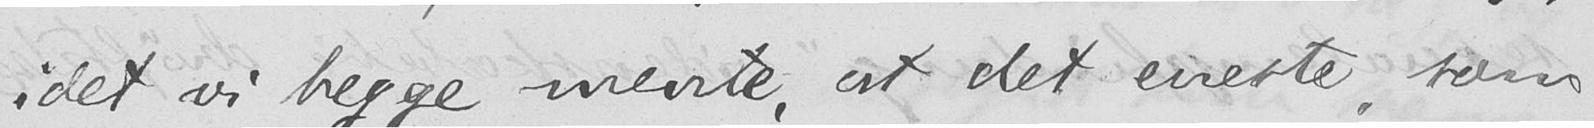idet vi begge mente, at det eneste som
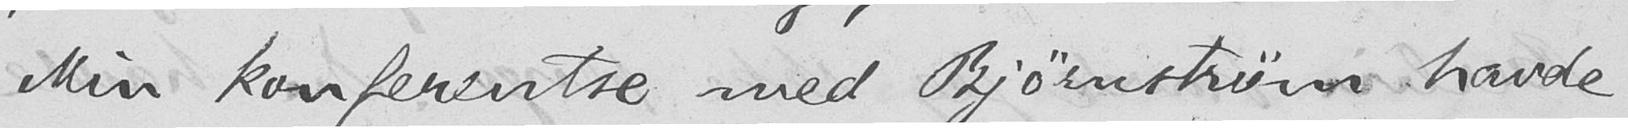Min konferentse med Bjørnstrøm havde
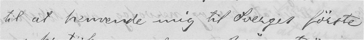til at henvende mig til Sveriges første
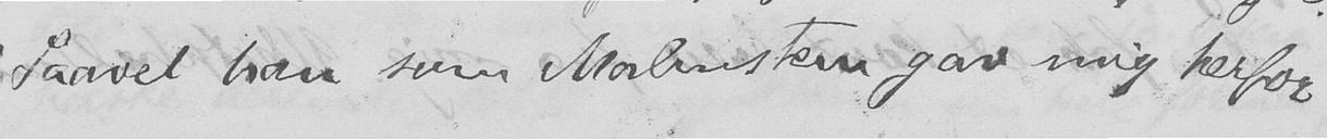Saavel han som Malmsten gav mig derfor
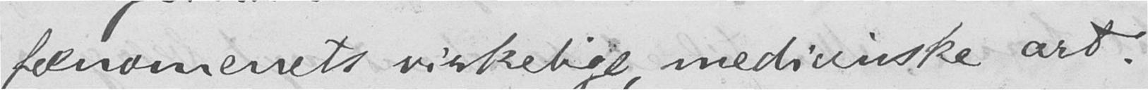fænomenets virkelige, medicinske art.
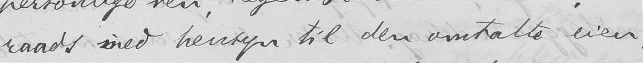raads med hensyn til den omtalte eien-
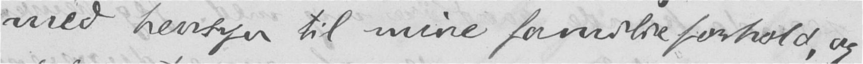med hensyn til mine familieforhold, og
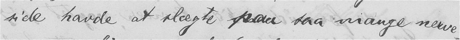side havde at slægte paa saa mange nerve-
